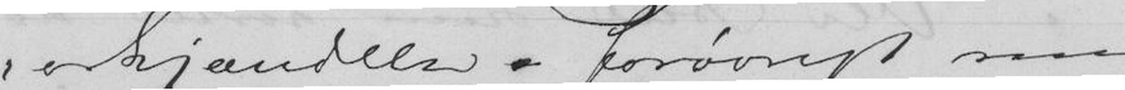erkjændelse. Forøvrigt maa
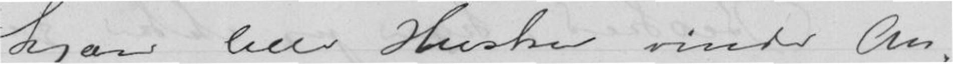kjære lille Hustru vinder An-
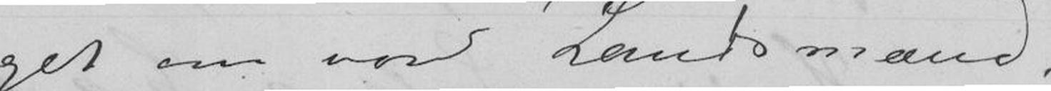get om vore Landsmænd.
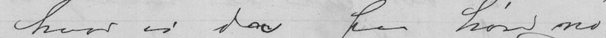hvor vi da faa høre no
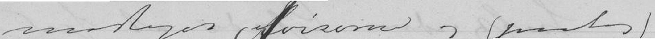modtaget Aviserne og (paatog)
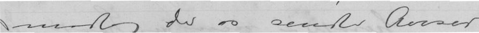modtog de os sendte Aviser
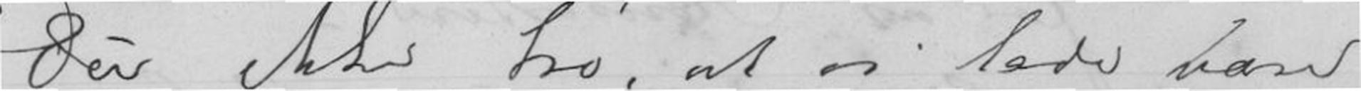Du ikke tro, at vi lade være
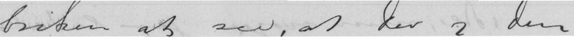briken at see, at du og din
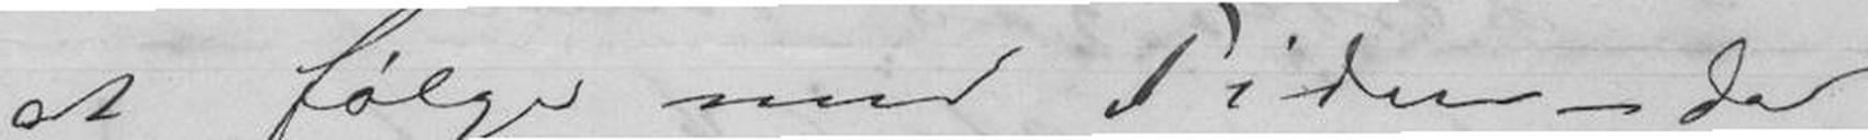at følge med Tiden - da
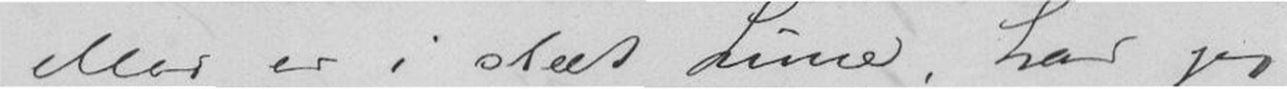eller er i sleet Lune, har jeg
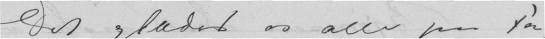Det glæder os alle paa Fa
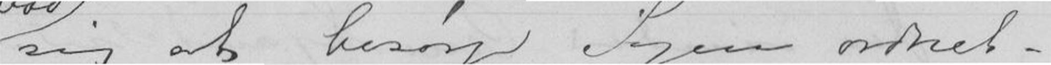sig at besørge Sagen ordnet -
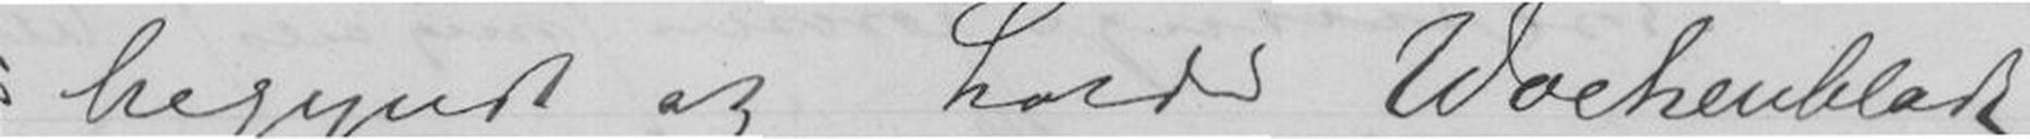begyndt at holde Wochenblatt
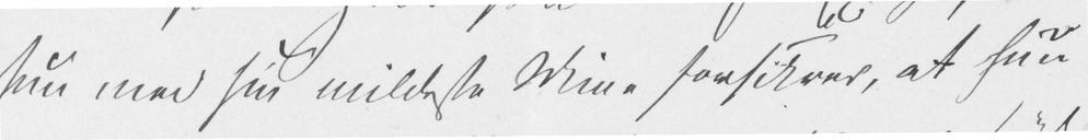hun med sin mildeste Mine forsikrer, at hun
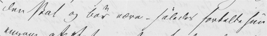den skal og bør være – således fortalte hun
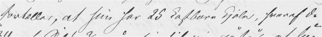fortæller, at hun har 25 kostbare Kjoler, hvoraf de
